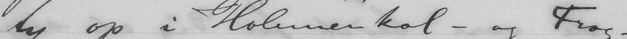ty op i Holmenkol- og Frog-
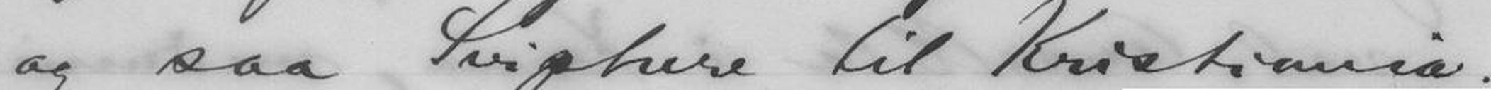og saa Svipture til Kristiania.
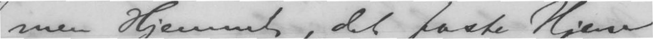men Hjemmet, det faste Hjem,
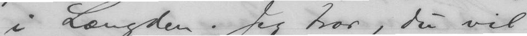i Længden. Jeg tror, Du vil
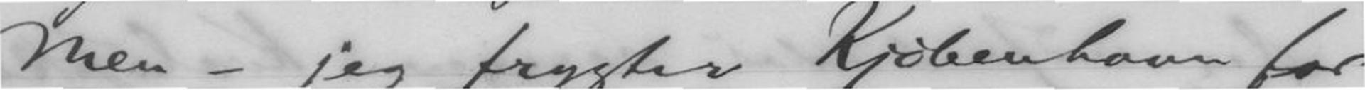Men - jeg frygter Kjøbenhavn for
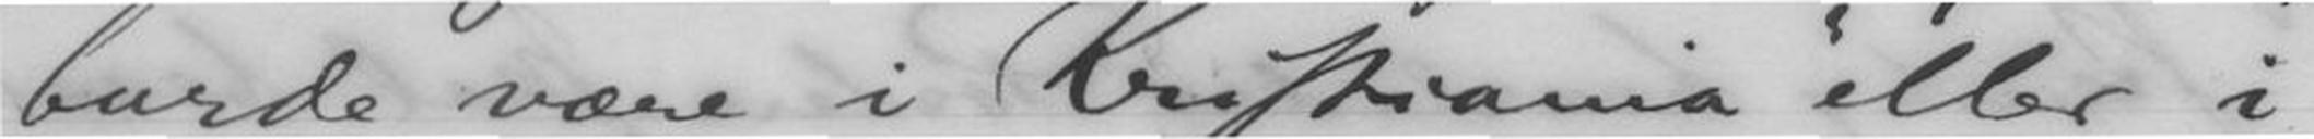burde være i Kristiania "eller i
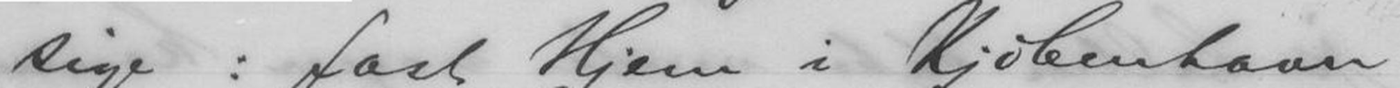sige: fast Hjem i Kjøbenhavn
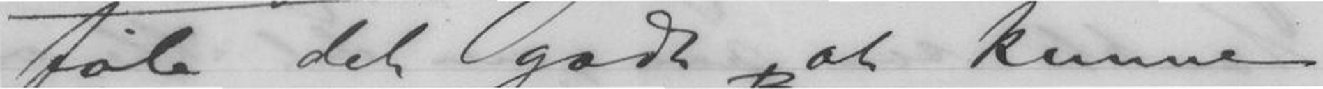føle det godt , at kunne
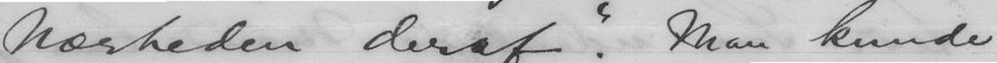Nærheden deraf". Man kunde
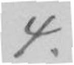4.
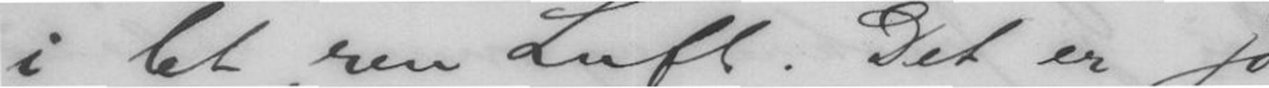i let, ren Luft. Det er jo
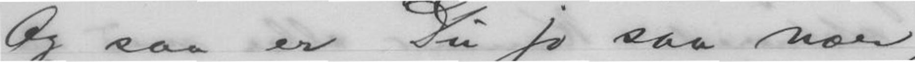Og saa er Du jo saa nær
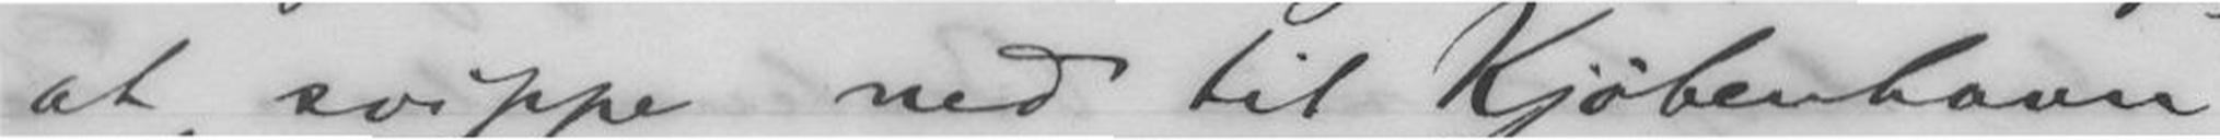at svippe ned til Kjøbenhavn
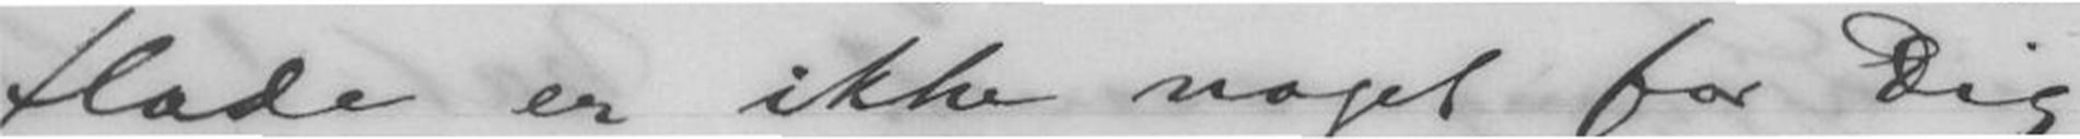flade er ikke noget for Dig
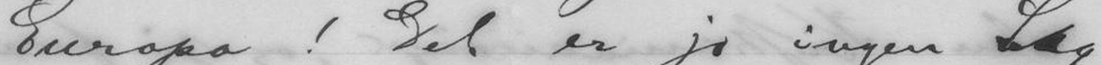Europa! Det er jo ingen Sag
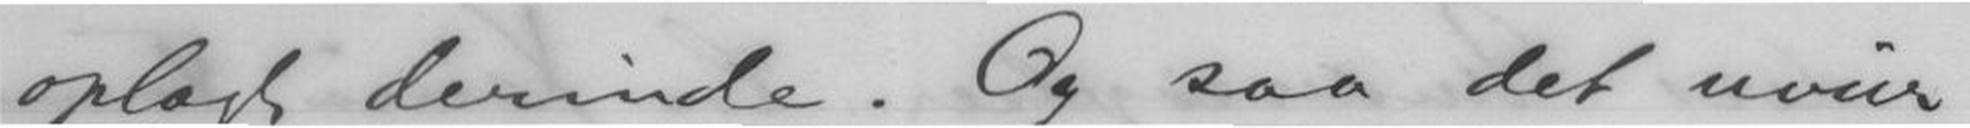oplagt derinde. Og saa det uvur-
